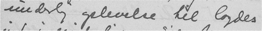underlig oplevelse til lagdes
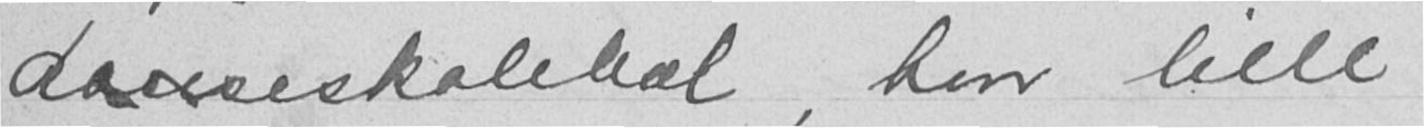danskeskolebal hvor lille
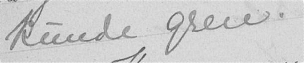kunde greie.
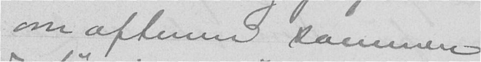om aftenen sammen
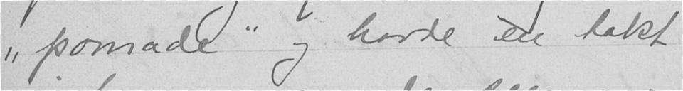"pomade" og hadde en takt
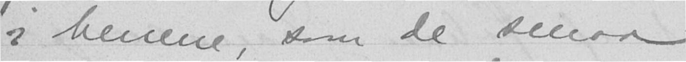i benene, som de smaa
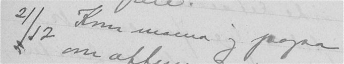21/12 Kom mama og papa
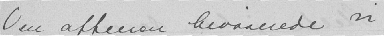Om aftenen bivaanede vi
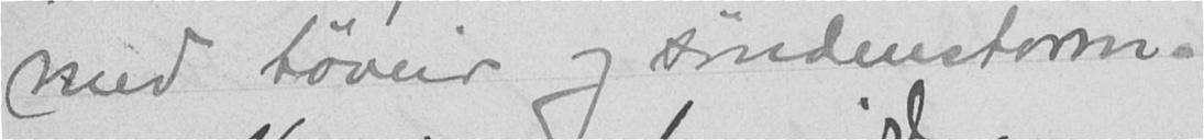med tøveir og søndenstorm.
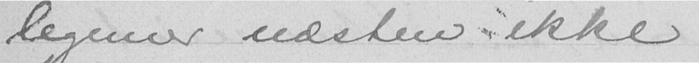legemer næsten ikke
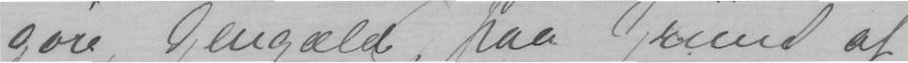gøre Gengæld paa Grund af
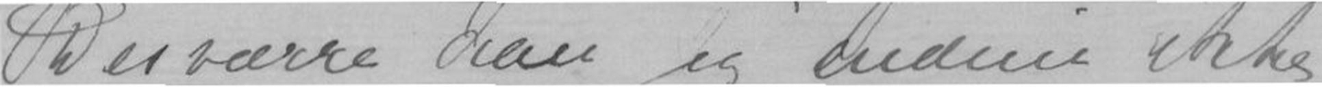Desværre kan jeg undnu ikke
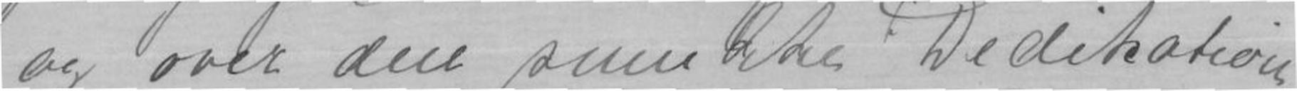og over den smukke Dedikation.
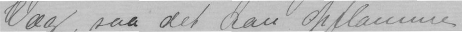Væg, saa det kan opflamme
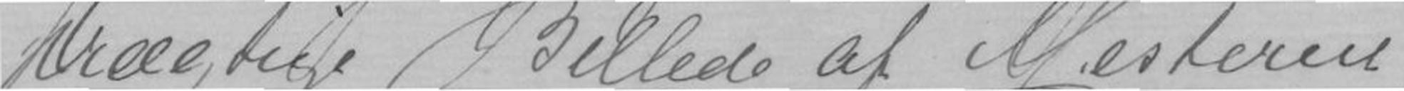prægtige Billede af Mesteren,
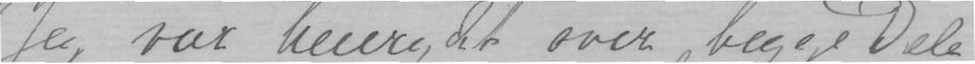Jeg var henrykt over begge Dele
